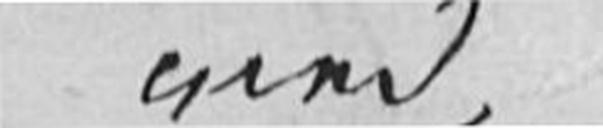med
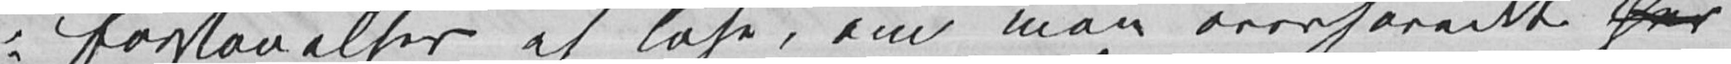forstaaelser at løse, om man overhovedet har
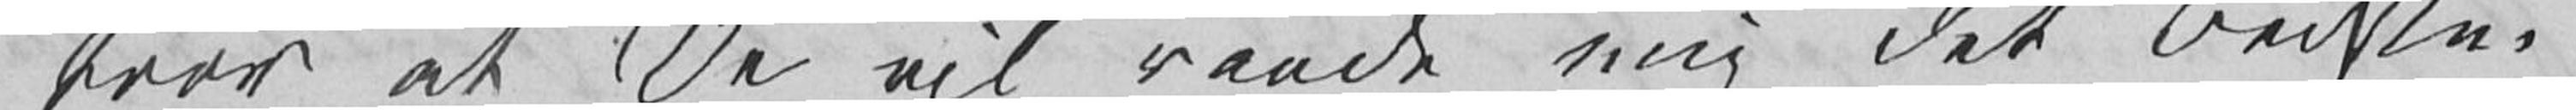tror at De vil raade mig det Bedste.
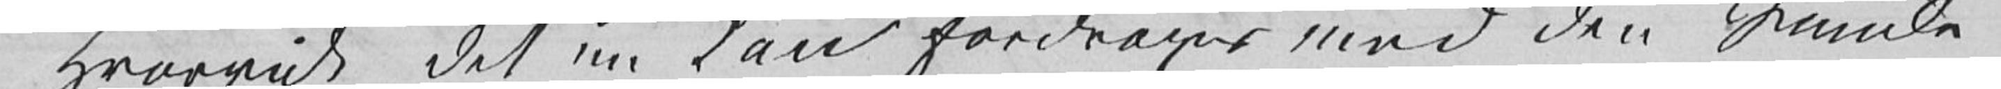Hvorvidt det nu kan fordrages med den Smule
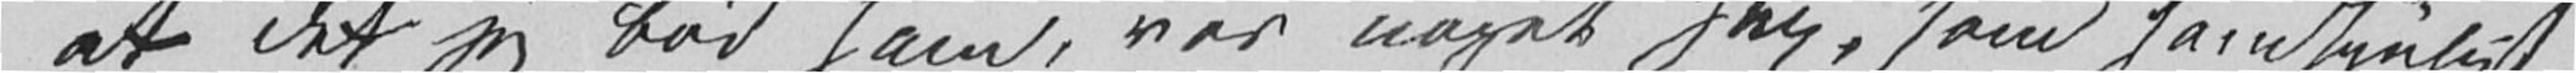at det jeg bød ham, var noget Jux, som sandsynligt
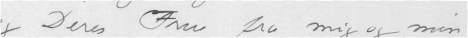og Deres Frue fra mig og min
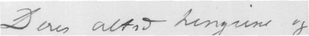Deres altid hengivne og
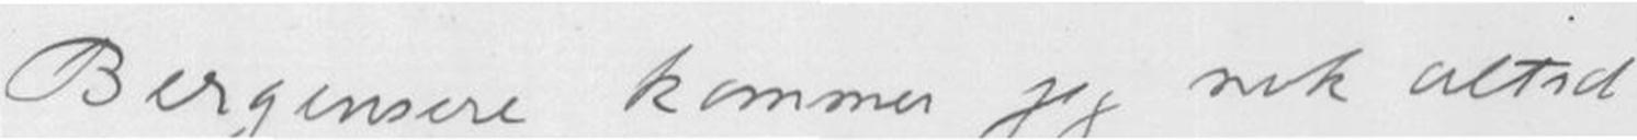Bergensere kommer jeg nok altid
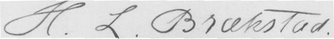H.L. Brækstad.
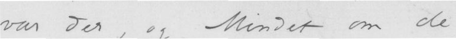var der, og Mindet om de
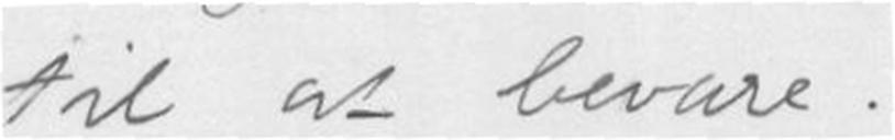til at bevare.
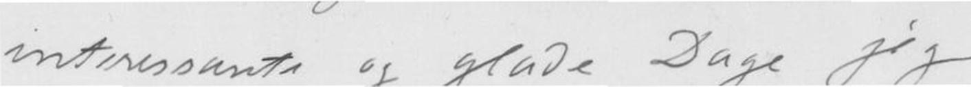interessante og glade Dage jeg
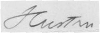Hustru
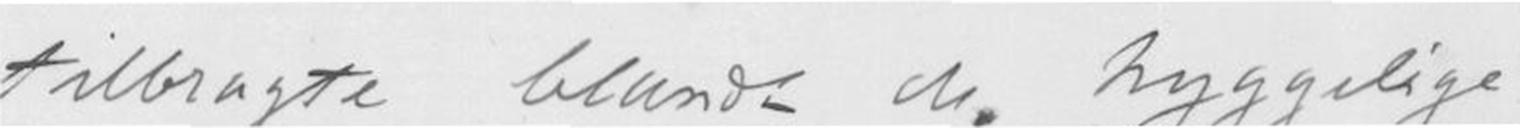tilbragte blandt de hyggelige
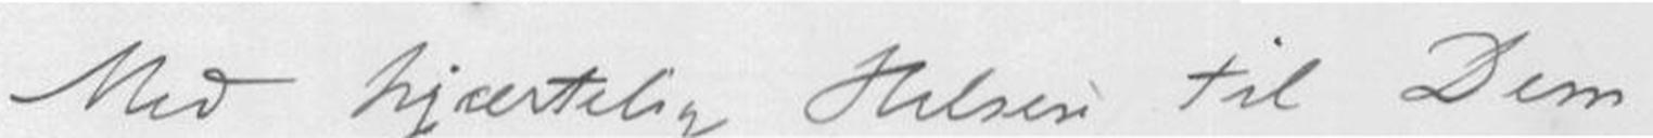Med hjertelig Hildsen til Dem
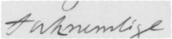taknemlige
